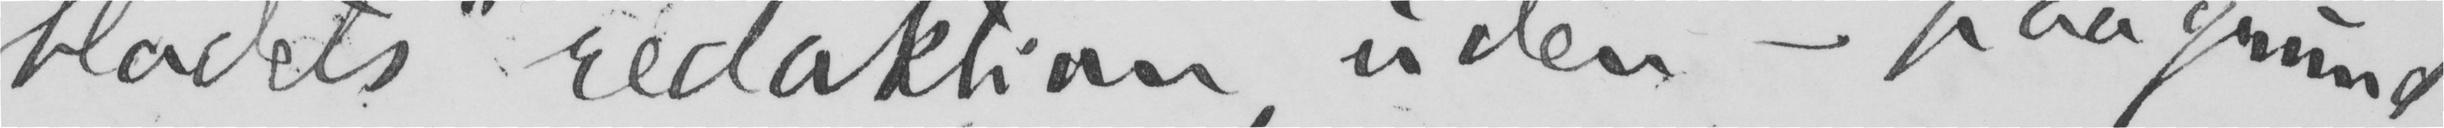bladets» redaktion, uden – paa grund
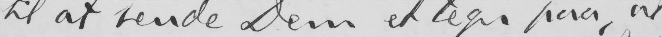til at sende Dem et tegn paa,
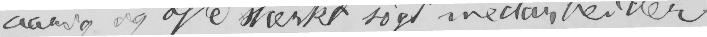aarig og ofte stærkt søgt medarbeider
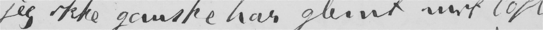at jeg ikke ganske har glemt mit løfte
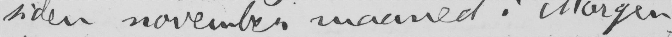siden november maaned i «Morgen-
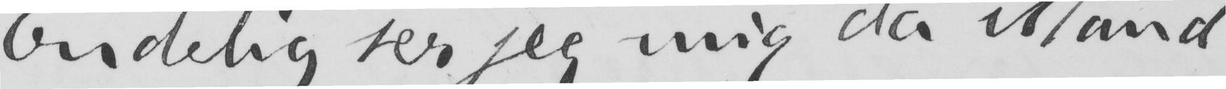Endelig ser jeg mig da istand
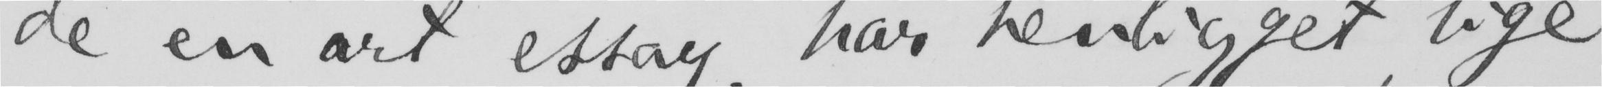de en art essay, har henligget lige
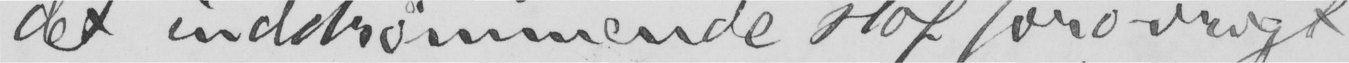det indstrømmende stof forøvrigt –
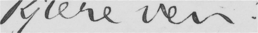Kjære ven!
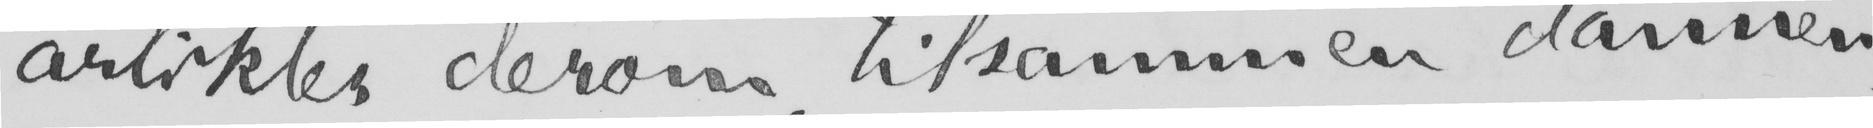artikler derom, tilsammen dannen-
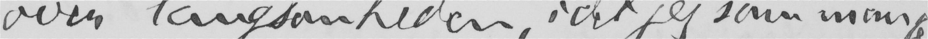over langdsomheden, idet jeg som mange-
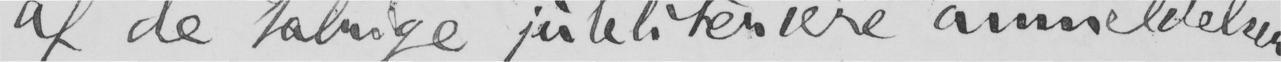af de talrige juleliterære anmeldelser og
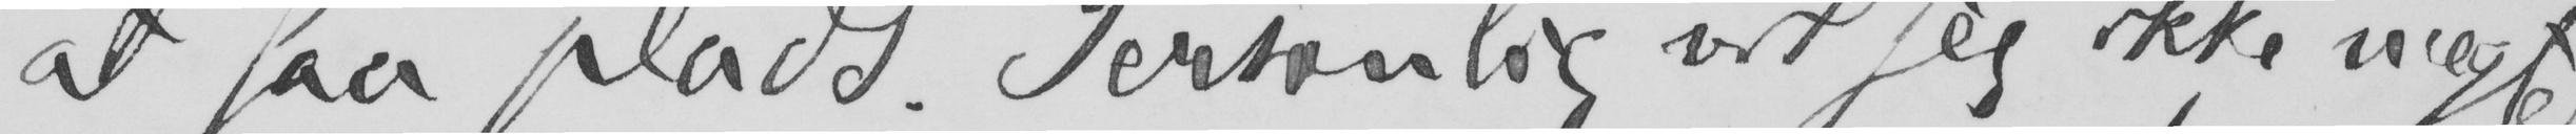at faa plads. Personlig vil jeg ikke nægte
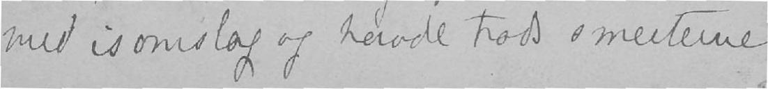med isomslag og havde trods smerterne
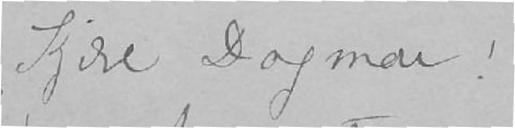Kjære Dagmar!
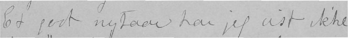Et godt nytaar har jeg vist ikke
